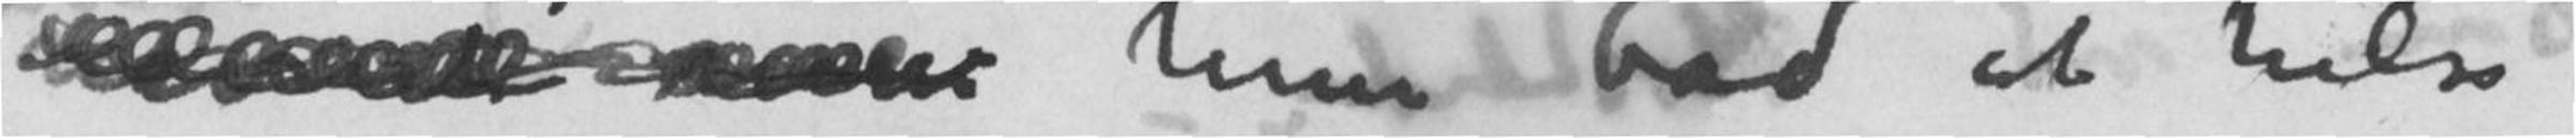hun bad at hilse
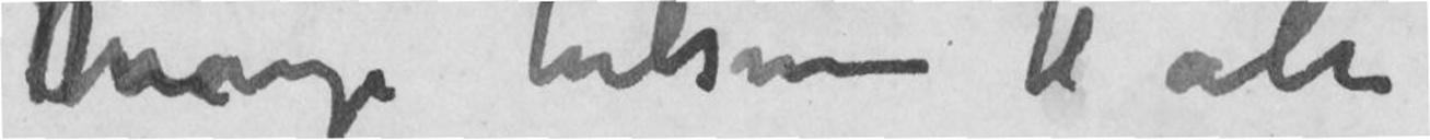Mange hilsener til alle
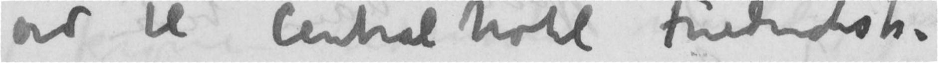ord til Central hotel Friedrichstr.
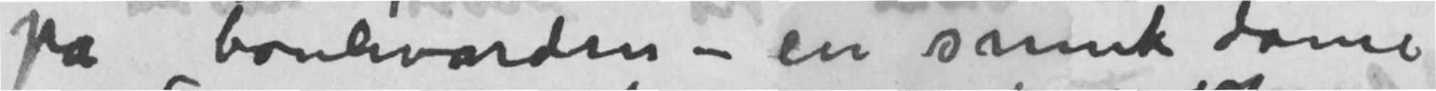på boulevarden – en smuk dame
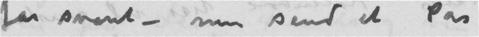får svaret – men send et Par
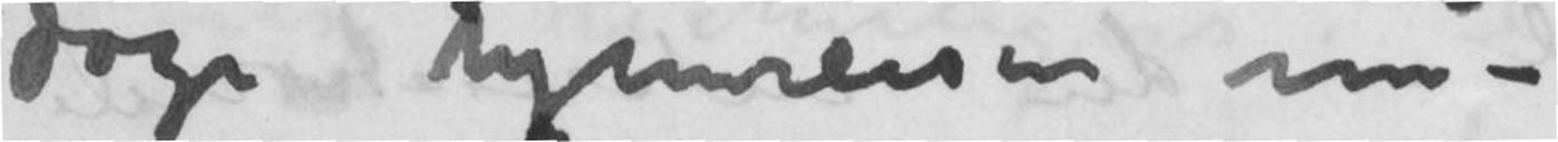dage hjemreisen nu –
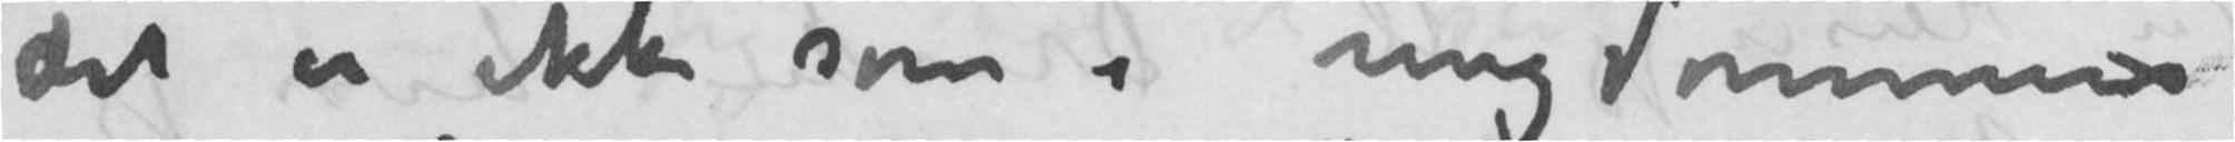det er ikke som i ungdommens
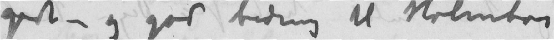godt – og god bedring til Holmboe
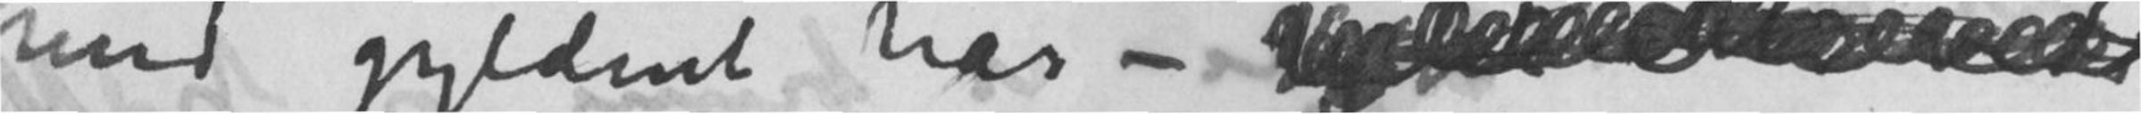med gyldent hår –
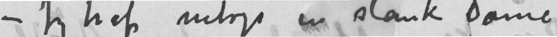– Jeg traf netop en slank dame
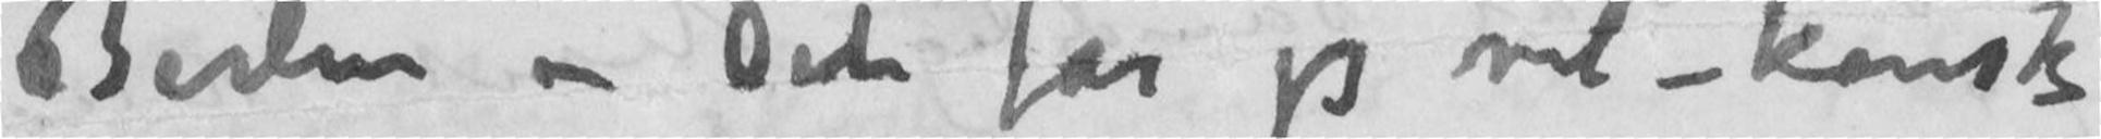Berlin – Det får jeg vel – kanske
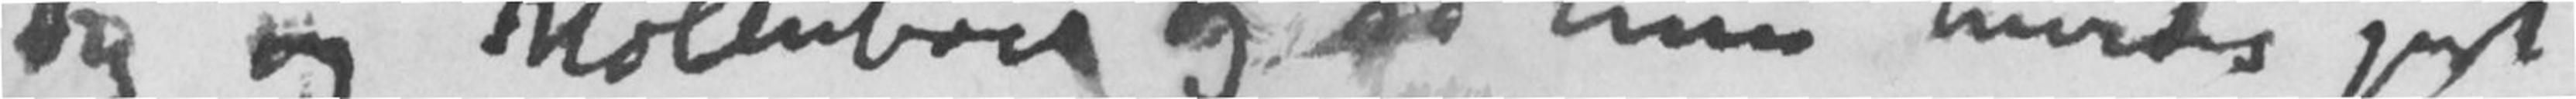Dig og Holmboes og si hun trivdes godt
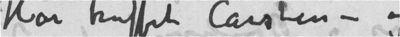Har truffet Carsten – og
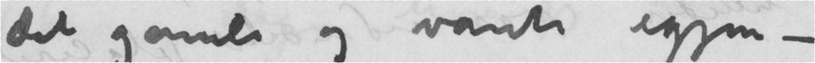det gamle og vante igjen –
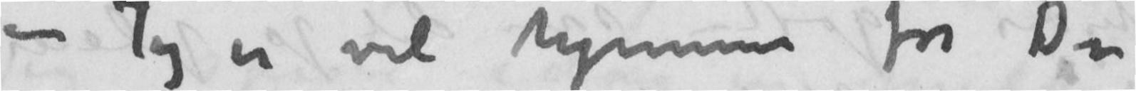– Jeg er vel hjemme før Du
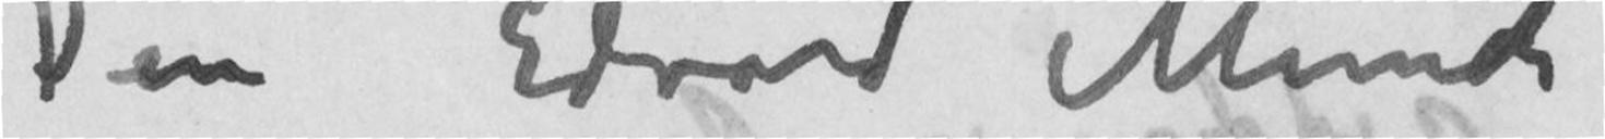Din Edvard Munch
